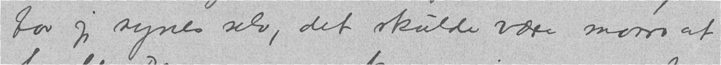for jeg synes selv, det skulde være morro at
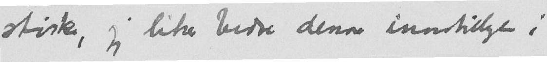stiske, jeg liker bedre denne innstillingen i
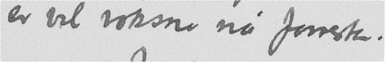er vel voksne nå forresten.
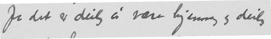Ja det er deilig av være hjemme og deilig
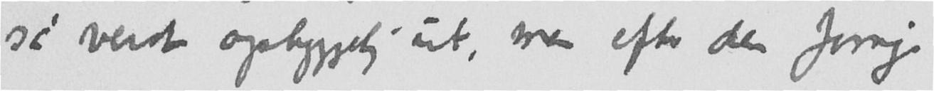så verst opbyggelig ut, men efter den forrige
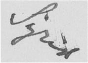Sigrid
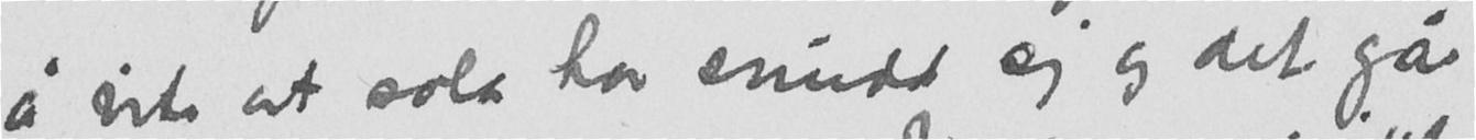å vite at sola har snudd sig og det går
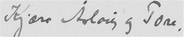Kjære Åslaug og Tore,
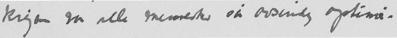krigen var alle mennesker så avsindig optimi-
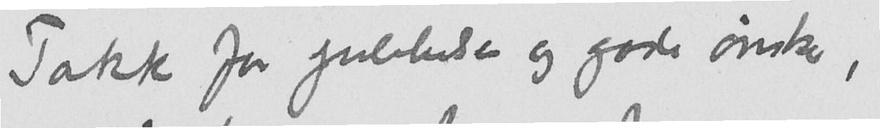Takk for julehilsen og gode ønsker,
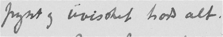frykt og uvisshet trods alt.
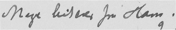Mange hilsener fra Hans,
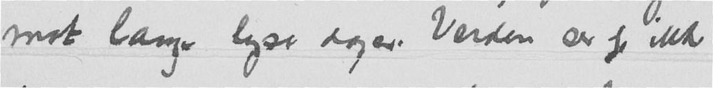mot langer lyse dager. Verden ser jo ikke
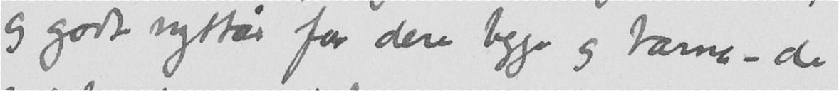og godt nyttår for dere begge og barna - de
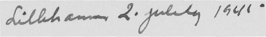Lillehammer 2. juledag 1945
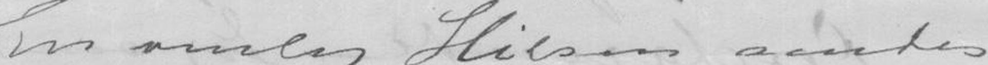En venlig Hilsen sendes
